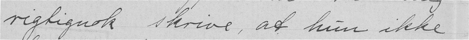rigtignok skrive, at hun ikke
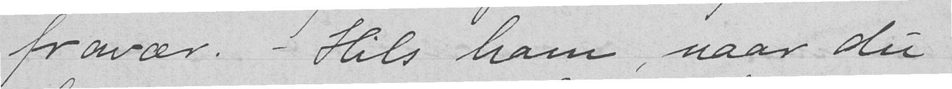fravær. - Hils ham, naar du
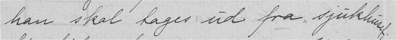han skal tages ud fra "sjukhuset",
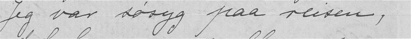Jeg var søsyg paa reisen,
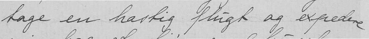tage en hastig flugt og expedere
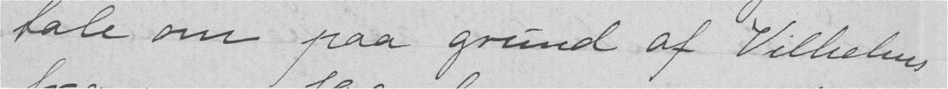tale om paa grund af Vilhelms
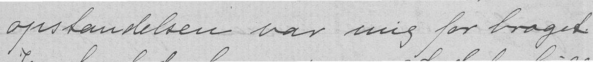opstandelsen var mig for broget.
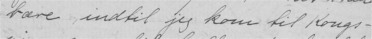bære, indtil jeg kom til Kongs-
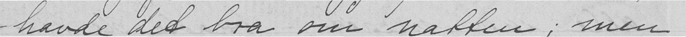havde det bra om natten; men
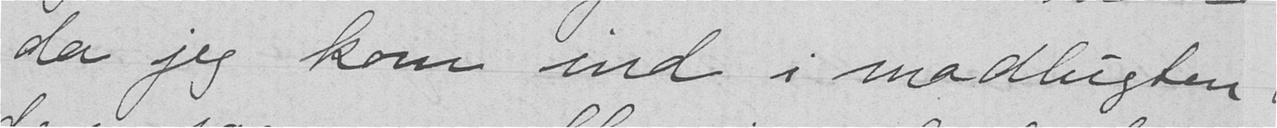da jeg kom ind i madlugten
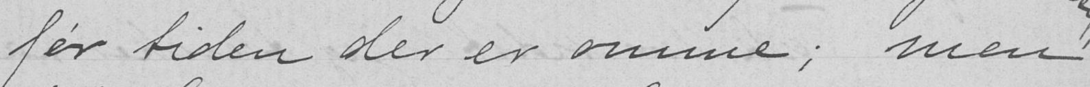før tiden der er omme; men
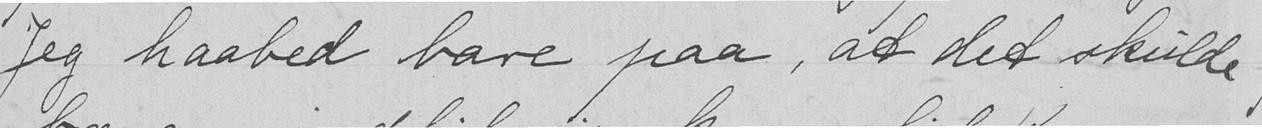Jeg haabed bare paa, at det skulde
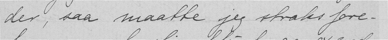der, saa maatte jeg straks fore-
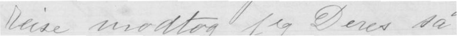reise modtog jeg Deres så
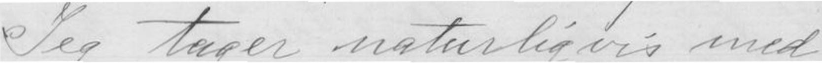Jeg tager naturligvis med
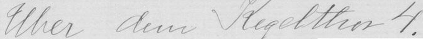Über den Kegelthon 4.
